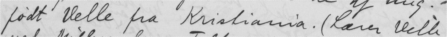født Velle fra Kristiania. (Lærer Velle
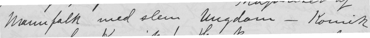Mannfolk med slem Ungdom - Komik
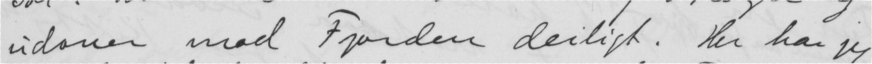udover mod Fjorden deiligt. Her har jeg
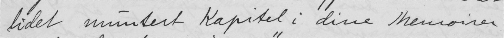lidet muntert Kapitel i dine Memoirer
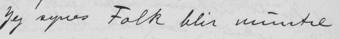Jeg synes Folk blir muntre
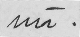nu.
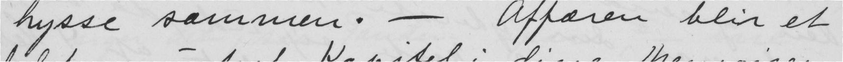hysse sammen. — Affæren blir et
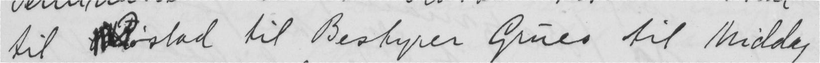til Røstad til Bestyrer Grues til Middag
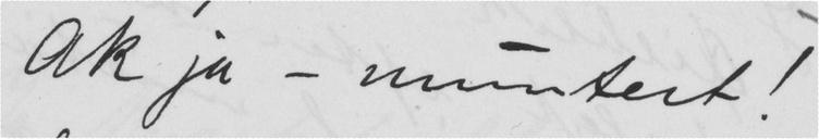Ak ja — muntert!
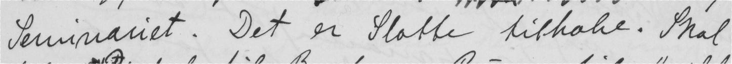Seminariet. Det er Slotte tilbake. Skal
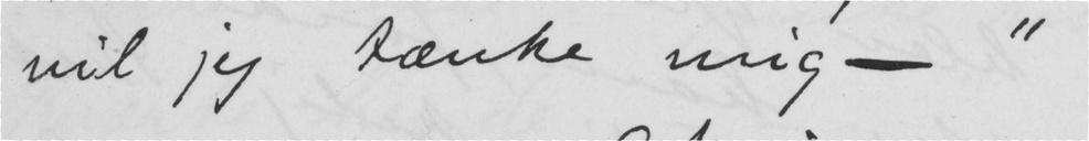til jeg tænke mig -"
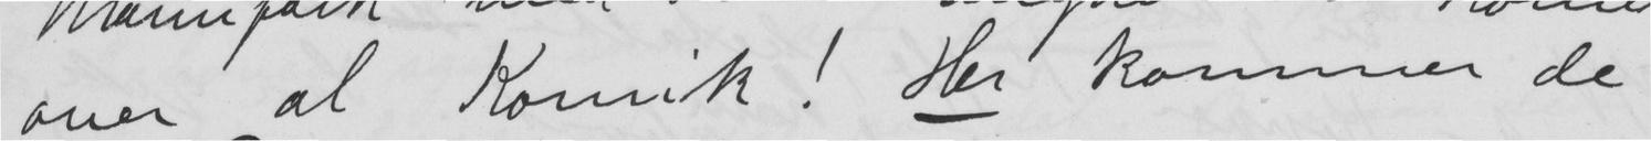over al Komik! Her kommer de
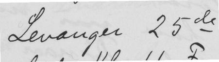Levanger 25de
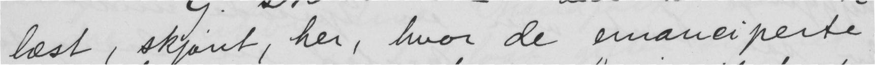læst, skjønt, her, hvor de emanciperte
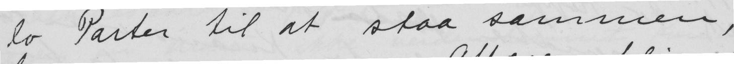to Parter til at staa sammen,
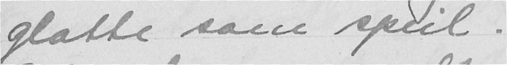glatte som speil.
